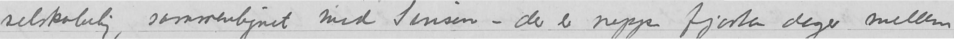selskabelig, sammenlignet med Sinsen – der er neppe fjorten dager mellem
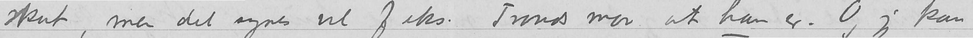skat, men det synes vel f eks. Tronds mor at han er. Og jeg kan
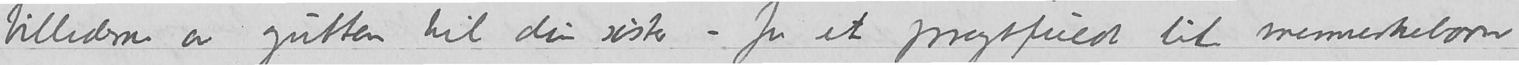billederne av gutten til din søster – for et pragtfuld lite menneskebarn
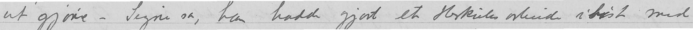at gjøre – Signe sa, han hadde gjort et Herkules arbeide i høst med
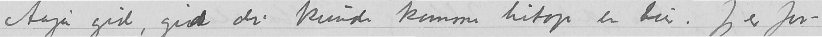Aaja gid, gid du kunde komme hitop en tur. Jeg er for-
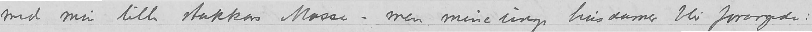med min lille stakkars Mosse – men mine unges husdamer blir forargede:
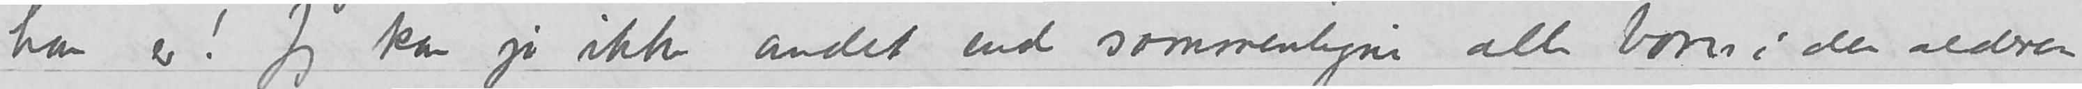han er! Jeg kan jo ikke andet end sammenligne alle barn i den alderen
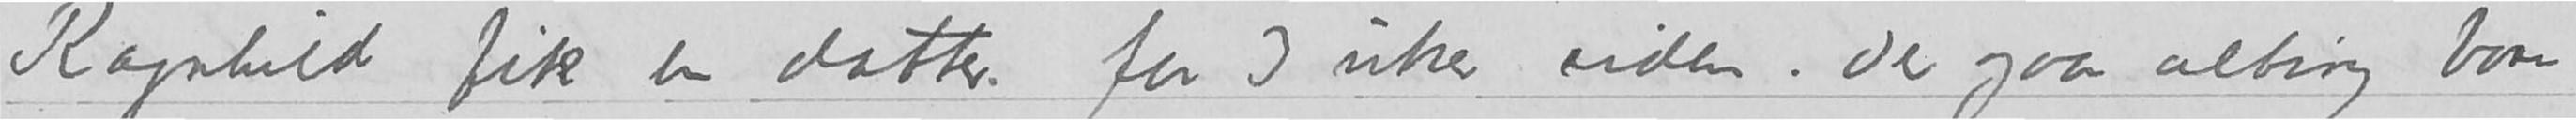Ragnhild fik en datter for 3 uker siden. Der gaar alting bare
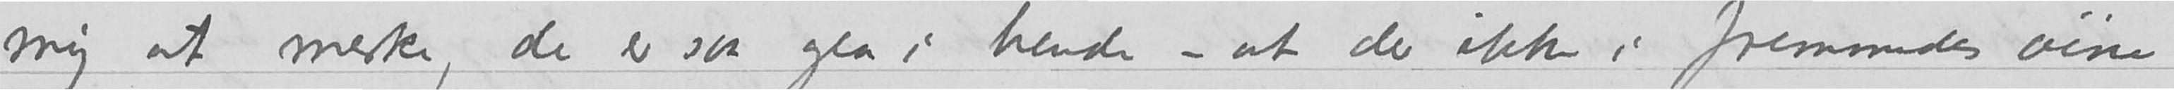mig at merke, de er saa gla i hende – at der ikke i fremmedes øine
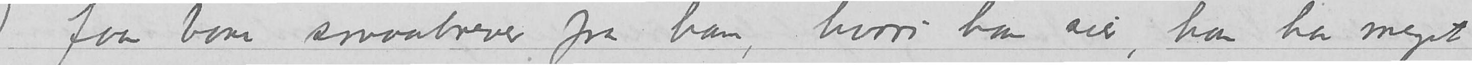Jeg faar bare smaabrever fra ham, hvori han sier, han har meget
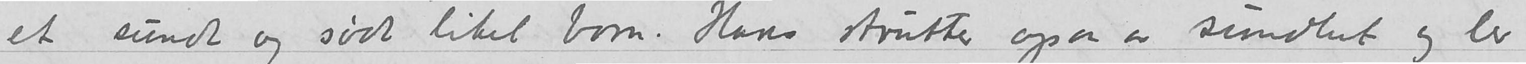et sundt og sødt litet barn. Hans strutter ogsaa av sundhet og
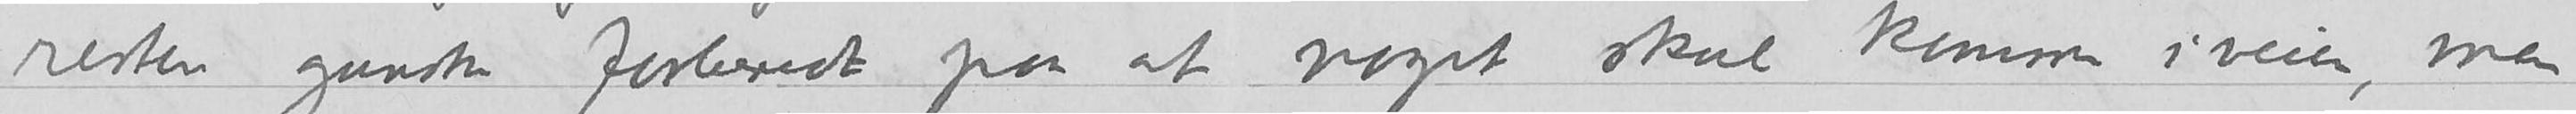resten ganske forberedt paa at noget skal komme iveien, men
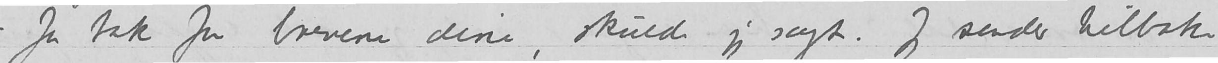–Ja tak for brevene dine, skulde jeg sagt. Jeg sender tilbake
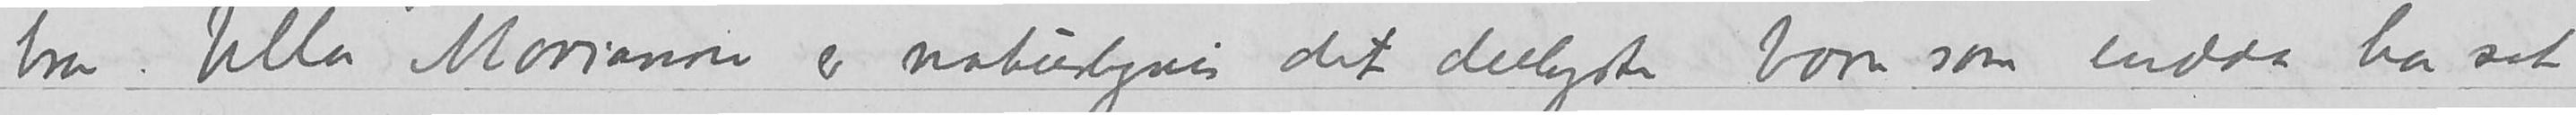bra. Ulla Marianne er naturligvis det deiligste barn som endda har set
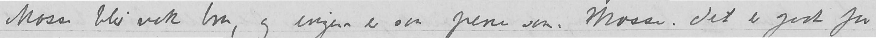Mosse blir nok bra, og ingen er saa pene som Mosse. Det er godt for
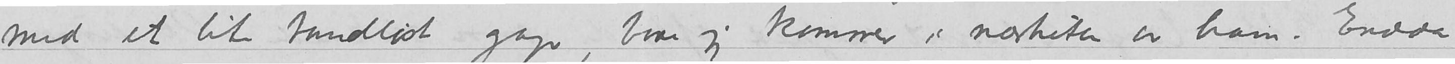ler med et lite tandløst gap, bare jeg kommer i nærheten av ham. Endda
